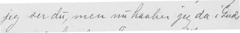jeg ser du, men nu haaber jeg da i Guds
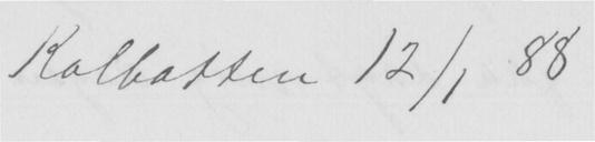Kolbotten 12/1 88
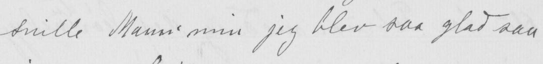snille Mann' min jeg blev saa glad saa
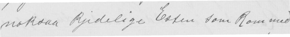noksaa kjedelige Ester som kom med
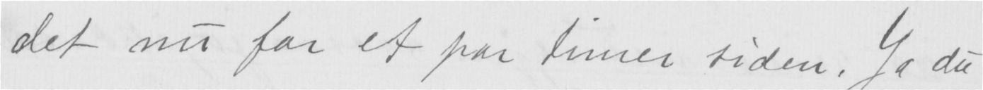det nu for et par timer siden. Ja du
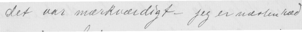det var mærkværdigt – jeg er næsten red
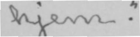hjem».
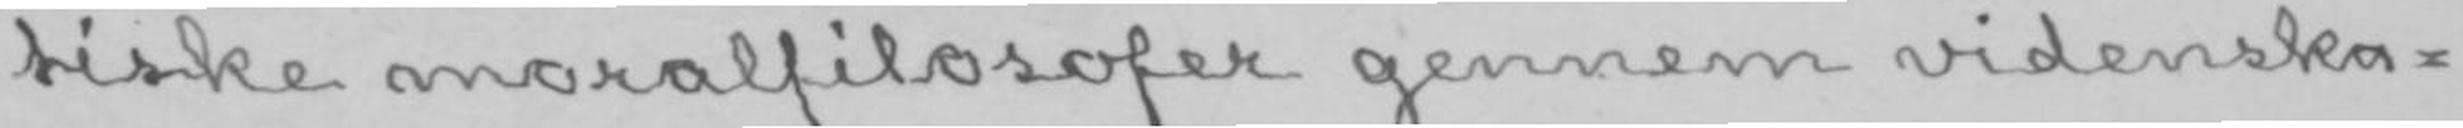tiske moralfilosofer gennem videnska-
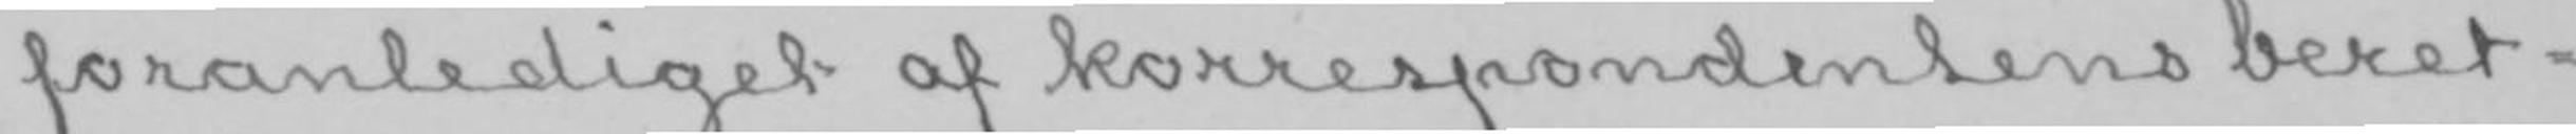foranlediget af korrespondentens beret-
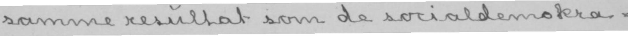samme resultat som de socialdemokra-
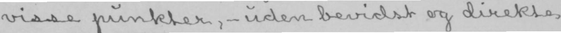visse punkter,- uden bevidst og direkte
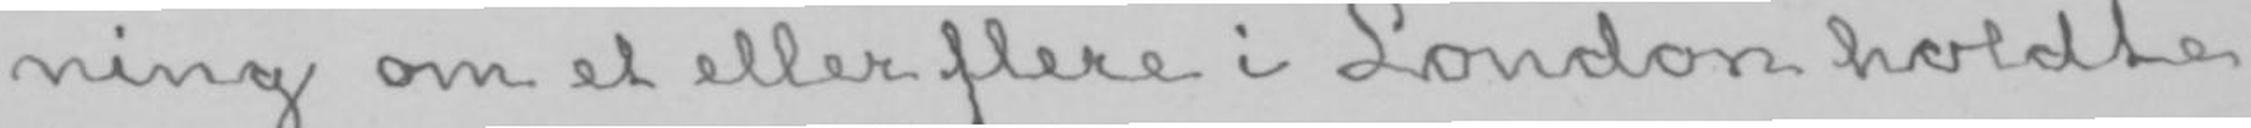ning om et eller flere i London holdte
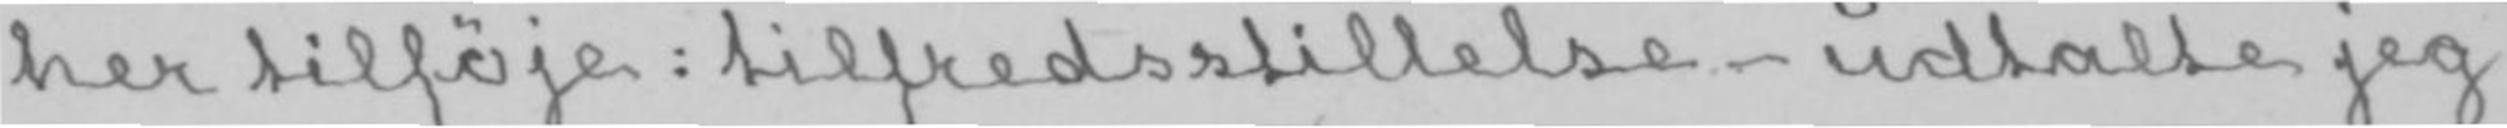her tilføje: tilfredsstillelse- udtalte jeg
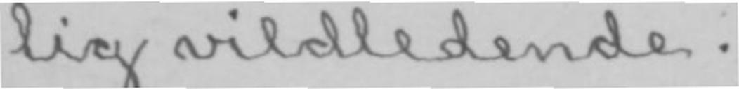lig vildledende.
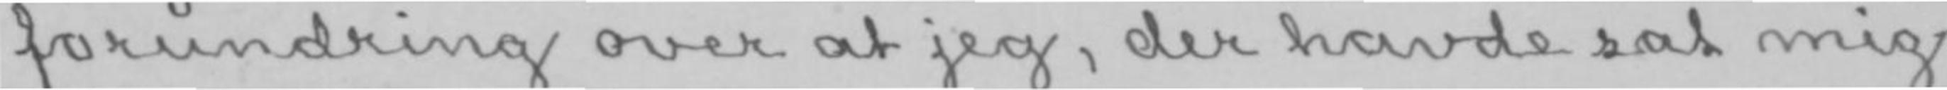forundring over at jeg, der havde sat mig
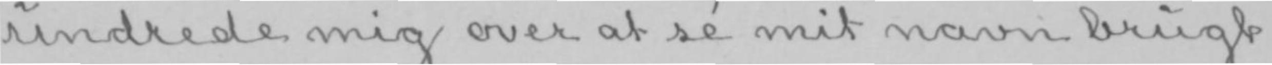undrede mig over at sé mit navn brugt
